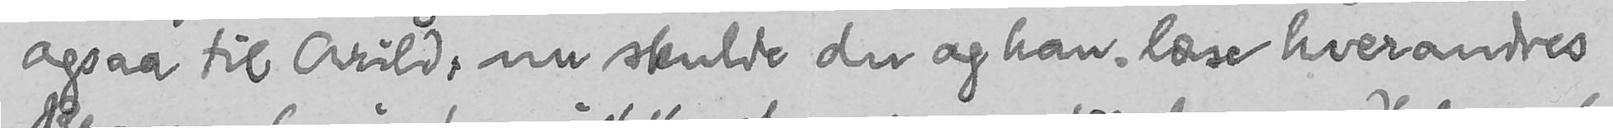ogsaa til Arild, nu skulde du og han læse hverandres
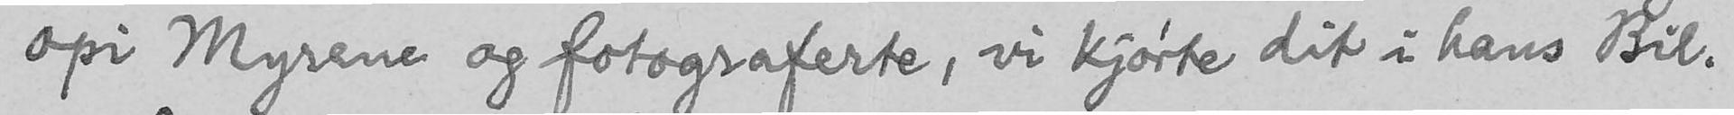opi Myrene og fotograferte, vi kjørte dit i hans Bil.
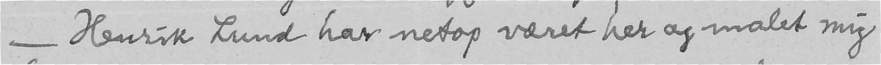- Henrik Lund har netop været her og malet mig
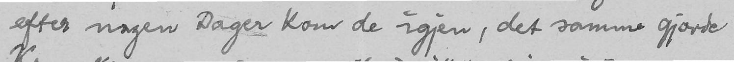efter nogen Dager kom de igjen, det samme gjorde
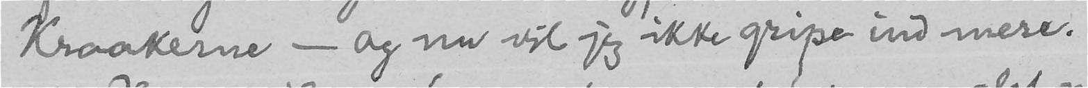Kraakerne - og nu vil jeg ikke gripe ind mere.
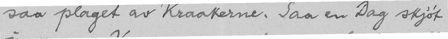saa plaget av Kraakerne. Saa en Dag skjøt
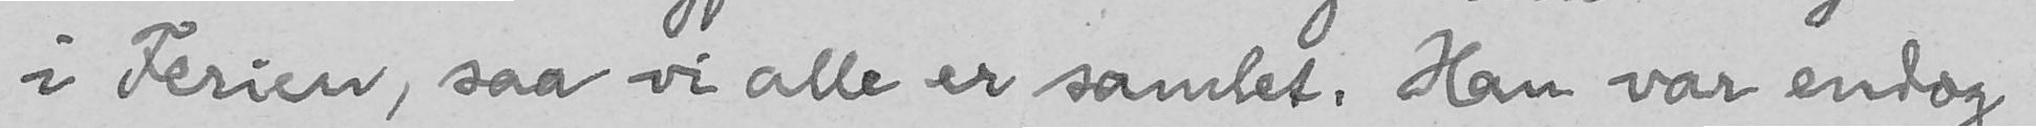i Ferien, saa vi alle er samlet. Han var endog
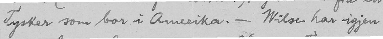Tysker som bor i Amerika. - Wilse har igjen
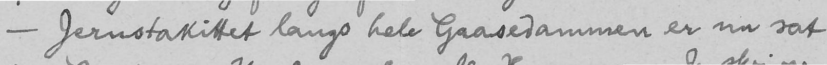- Jernstakittet langs hele Gaasedammen er nu sat
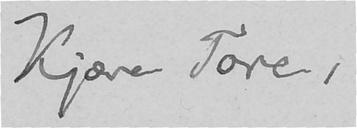Kjære Tore,
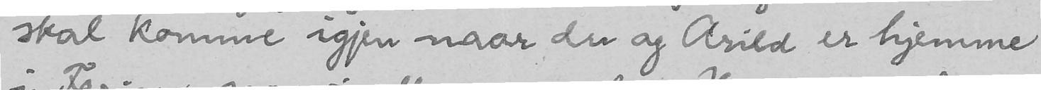skal komme igjen naar du og Arild er hjemme
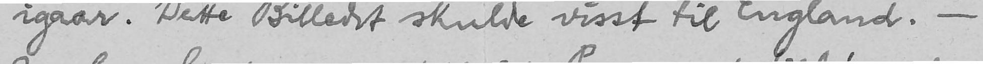igaar. Dette Billedet skulde visst til England. -
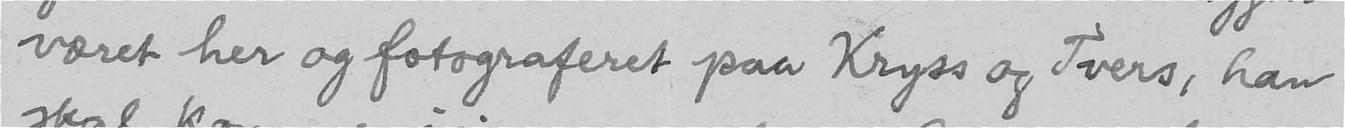været her og fotograferet paa Kryss og Tvers, han
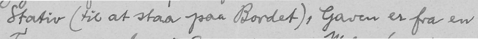Stativ (til at staa paa Bordet), Gaven er fra en
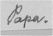Papa.
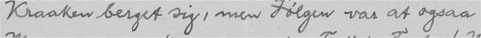Kraaken berget sig, men Følgen var at ogsaa
